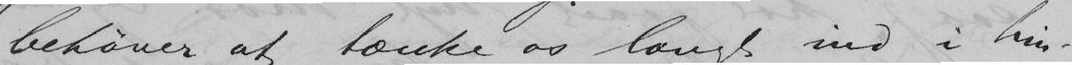behøver at tænke langt ind i hin-
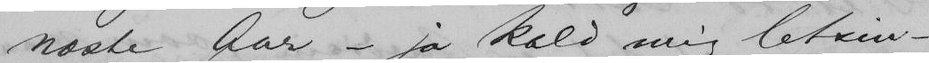næste Aar - ja kald mig letsin-
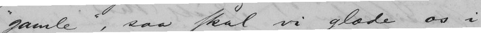"gamle", saa skal vi glæde os i
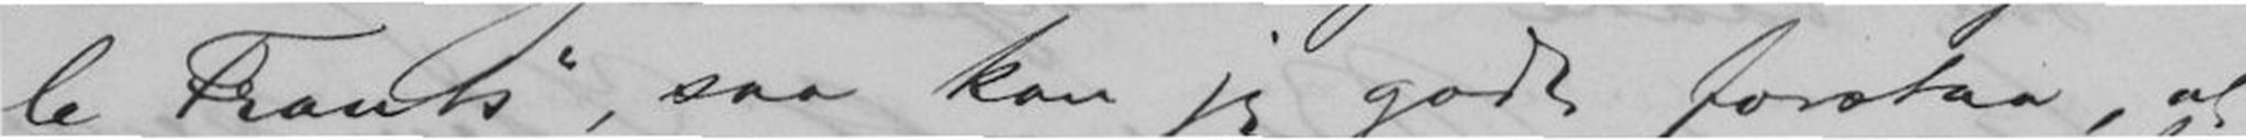le Frants", saa kan jeg godt forstaa, at
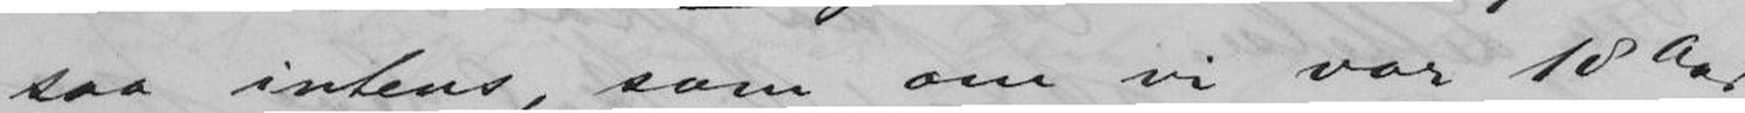saa intens, som om vi var 18 Aar.
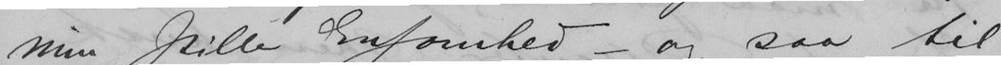min stille Ensomhed - og saa til
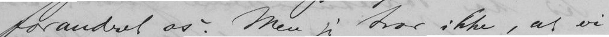forandret os. Men jeg tror ikke, at vi
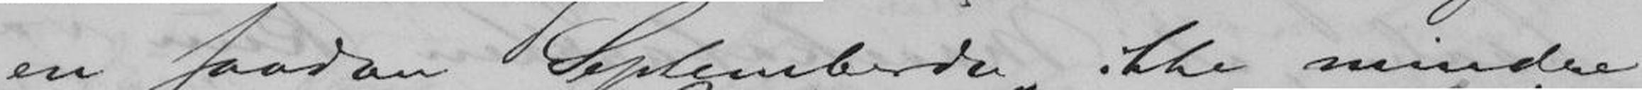en saadan Septemberdag ikke mindre
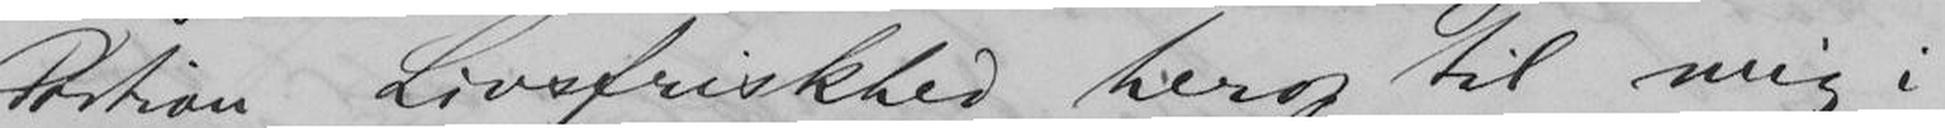Portion Livsfriskhed herop til mig i
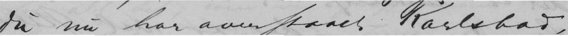Du nu har ovenstaaet Karlsbad,
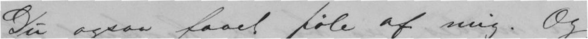Du ogsaa faaet føle af mig. Og
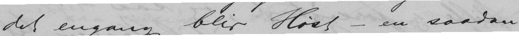det engang blir Høst - en saadan
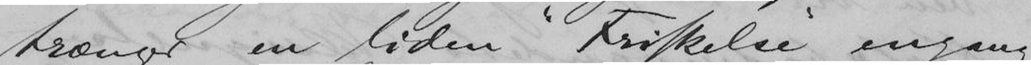trænger en liden "Friskelse" engang
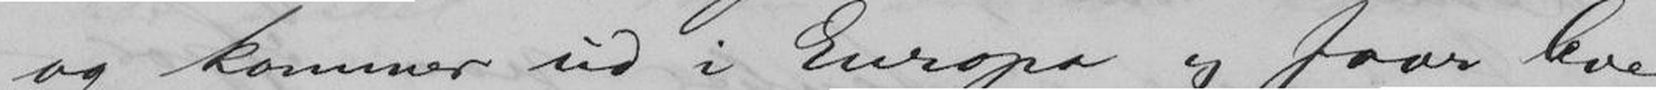og kommer ud i Europa og faar leve
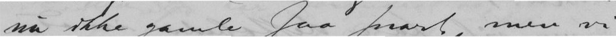nu ikke gamle saa snart, men vi
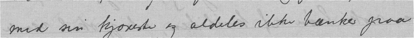med sin kjæreste og aldeles ikke tænker paa
